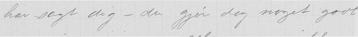har sagt dig - du gjør dog noget godt
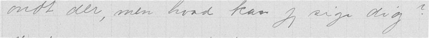ondt der, men hvad kan jeg sige dig?
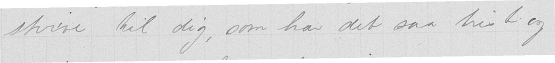skrive til dig, som har det saa trist og
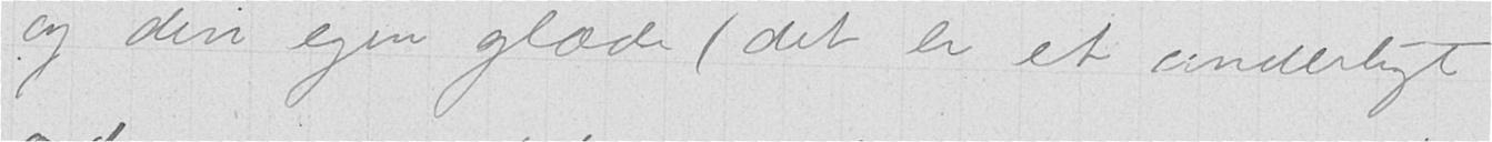og din egen glæde (det er et underligt
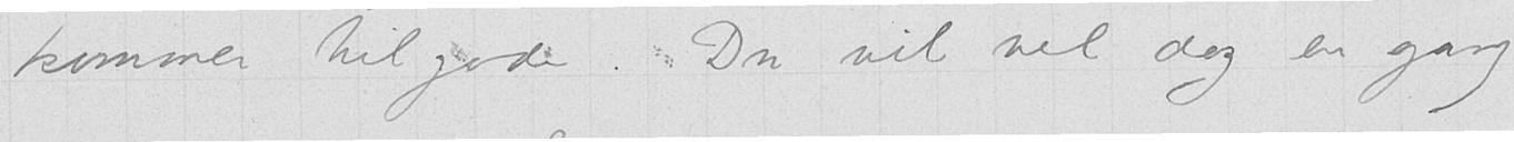kommer til gode. Du vil vel dog en gang
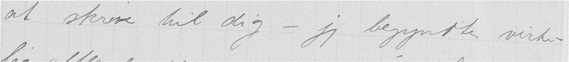at skrive til dig - jeg begynte virke-
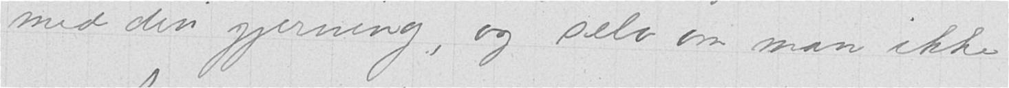med din gjerning, og selv om man ikke
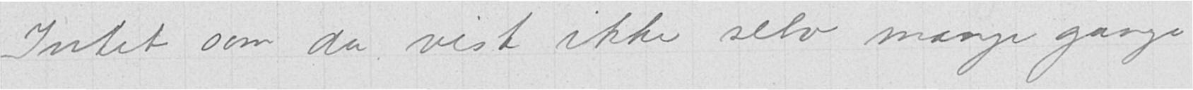Intet som du vist ikke selv mange gange
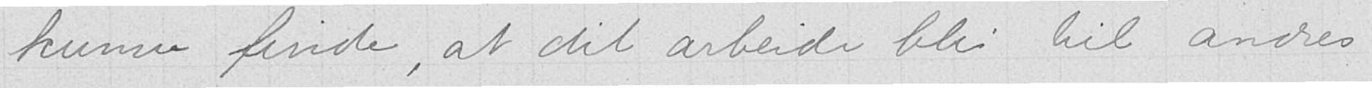kunne finde, at dit arbeide blir til andres
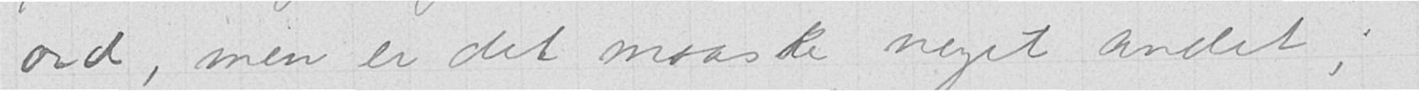ord, men er det maaske noget andet;
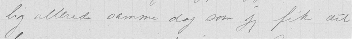lig allerede samme dag som jeg fik dit
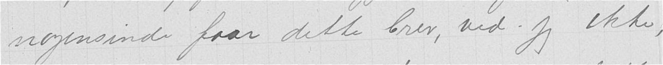nogensinde faar dette brev, ved jeg ikke,
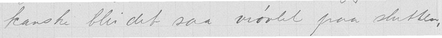kanske blir det saa mørkt paa slutten,
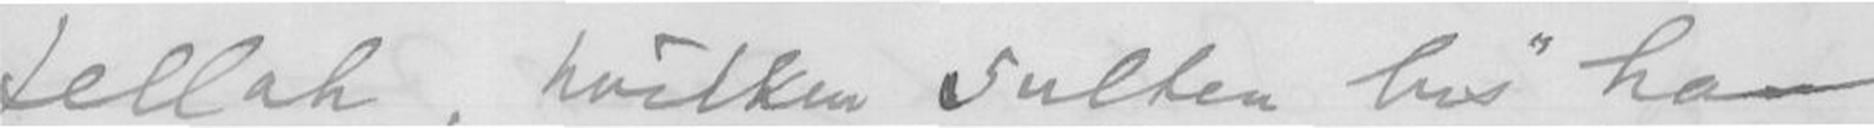"fellah, hvilken sulten bes" han
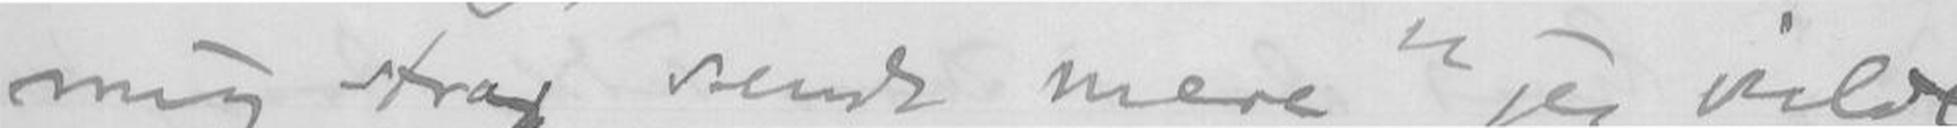mig strax sende mere "jeg vilde
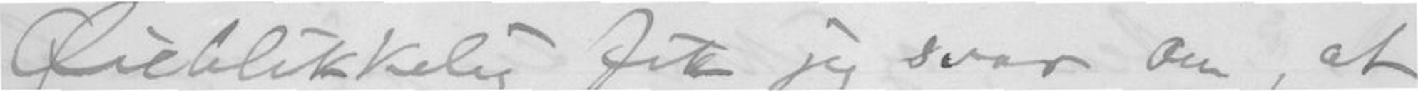Øieblikkelig fik jeg svar om, at
